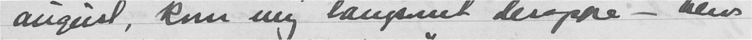august, kom mig langsomt deroppe - blev
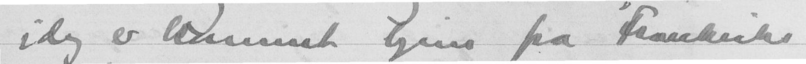idag er kommet hjem fra Frankirke
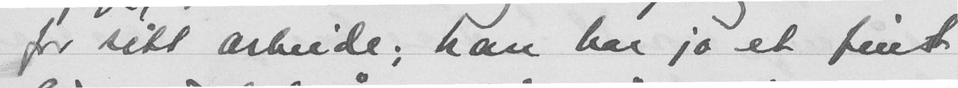for sitt arbeide, han har jo et fint
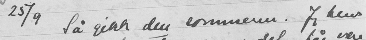25/9 Så gikk den sommeren. Jeg blev
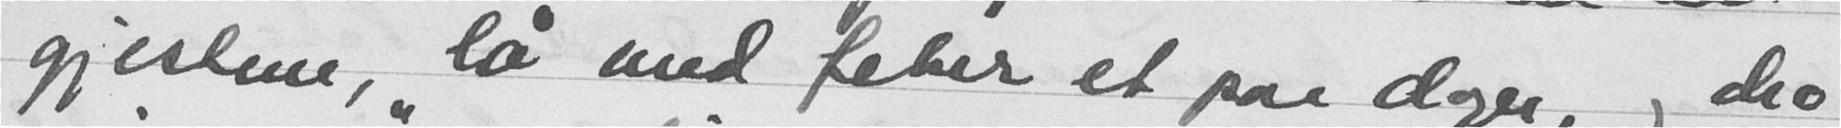gjestene, lå med feber et par dager, og dro
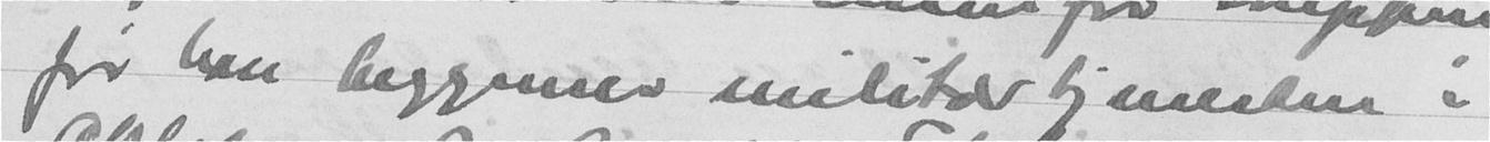før han begynner militærtjenesten i
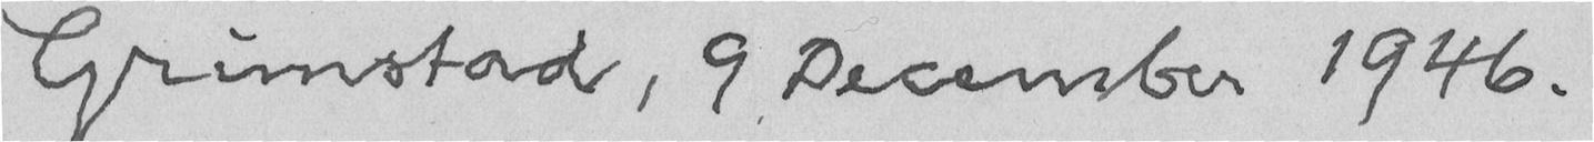Grimstad, 9 December 1946.
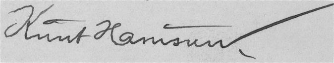Knut Hamsun.
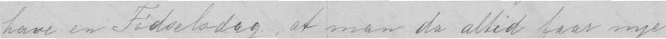have en Fødselsdag at man da altid faar nye
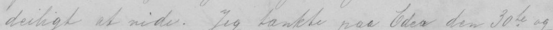deiligt at vide. jeg tænkte paa Eders den 30te og
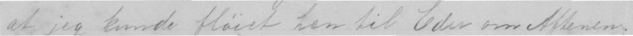at jeg kunde fløvet hen til Eder om Aftenen;
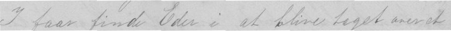I faar finde Eder i at blive taget over at
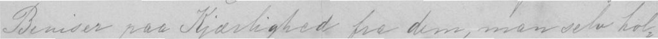Beviser paa Kjærlighed fra dem, men selv hol-
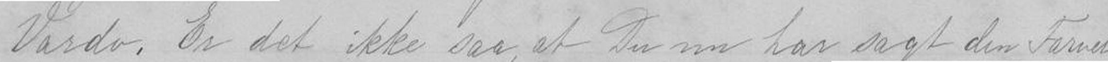Vardo. Er det ikke saa, at Du no har sagt dem Farvel
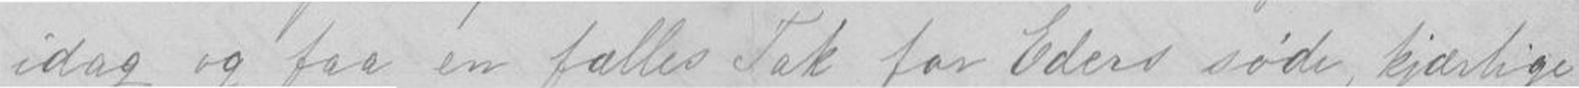idag ig faa en fælles Tak for Eders søde, kjærlige
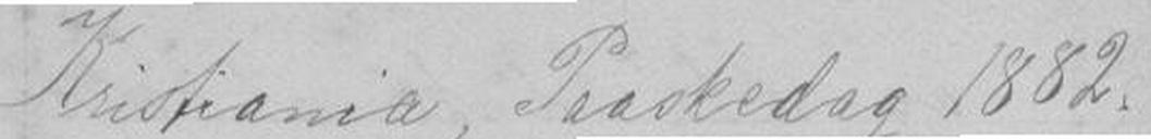Kristianin, Paaskedag 1882.
